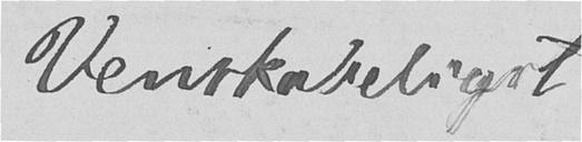Venskabeligst
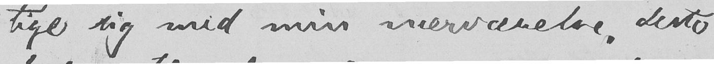tige sig med min nærværelse, desto
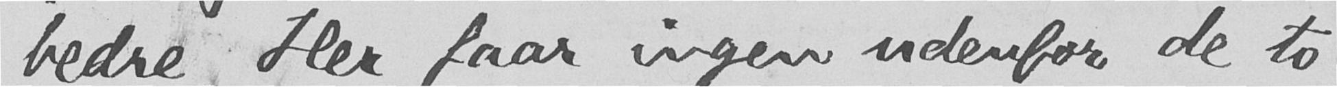bedre. Her faar ingen udenfor de to
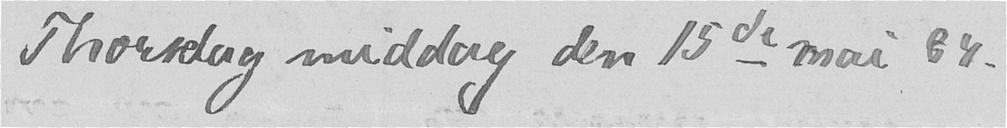Thorsdag middag den 15de mai 84.
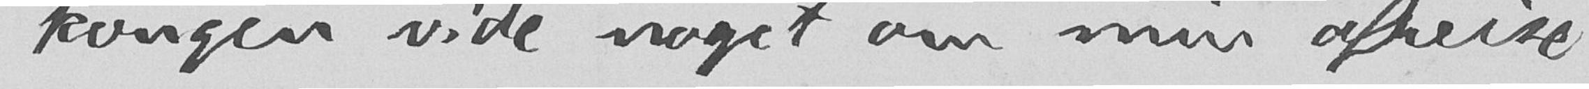kongen vide noget om min afreise.
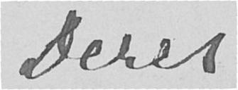Deres
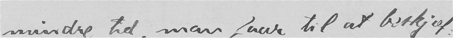mindre tid, man faar til at beskjæf-
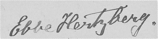Ebbe Hertzberg.
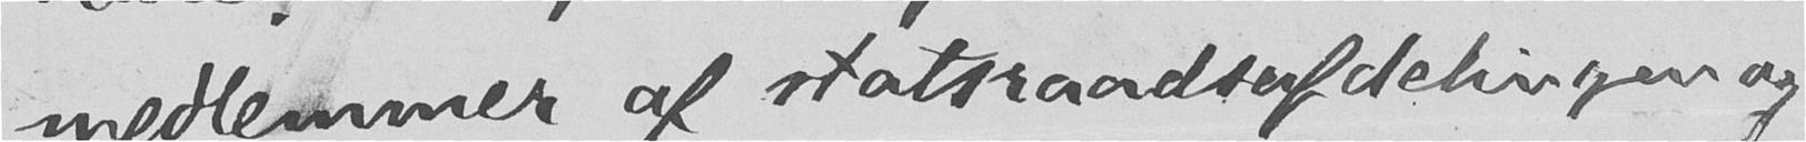medlemmer af statsraadsafdelingen og
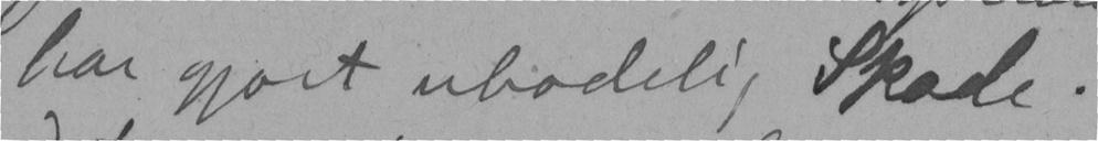har gjort ubødelig Skade.
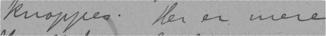knoppes. Her er mere
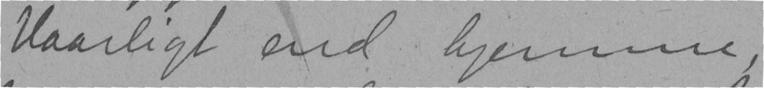Vaarligt end hjemme,
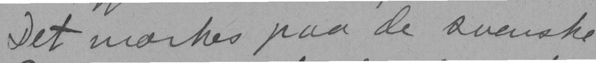Det mærkes paa de svenske
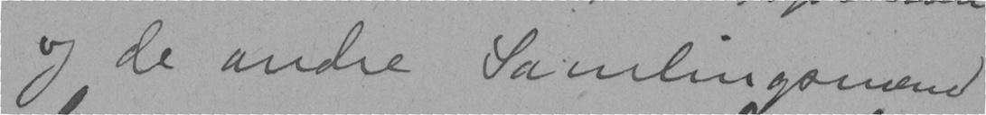og de andre Samlingsmend
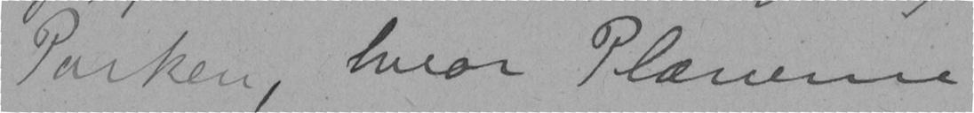Parken, hvor Plænerne
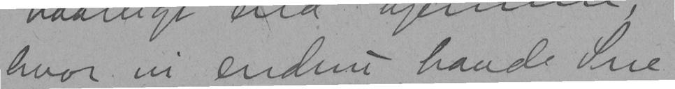hvor vi endnu havde Sne
